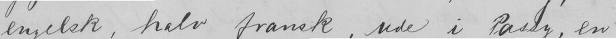engelsk, halv fransk, ude i Passy, en
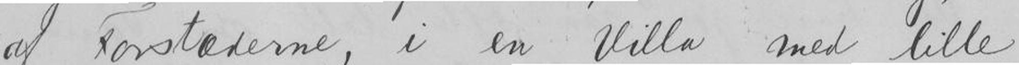af Forstæderne, i en Villa med lille
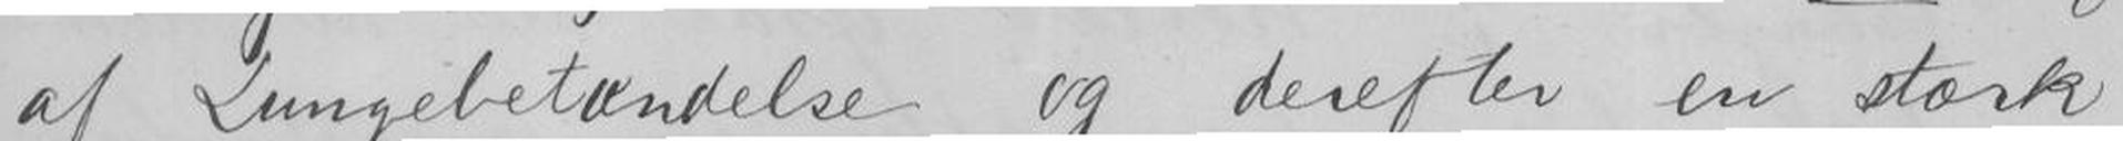af Lungebetændelse og derefter en stærk
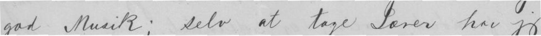god musik; selv at tage Lærer har jeg
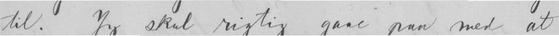til. Jeg skal rigtig gaae paa med at
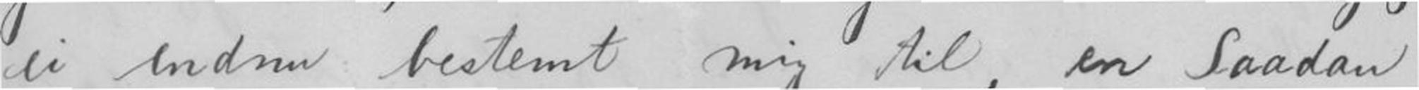ei endnu bestemt mig til, en Saadan
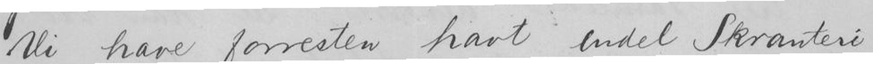Vi have forresten havt endel Skranteri
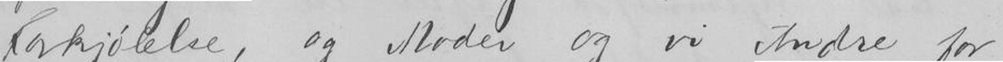forkjølelse, og Moder og vi Andre for
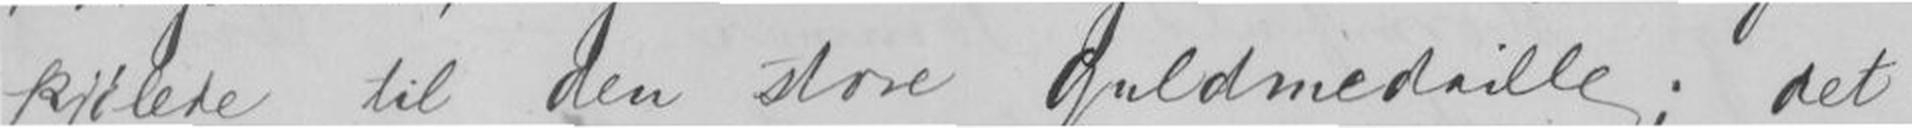kølede til den store guldmedaille; det
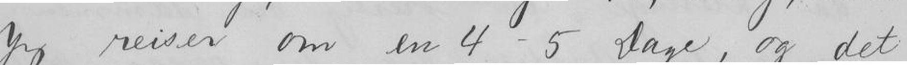Jeg reiser om en 4-5 Dage, og det
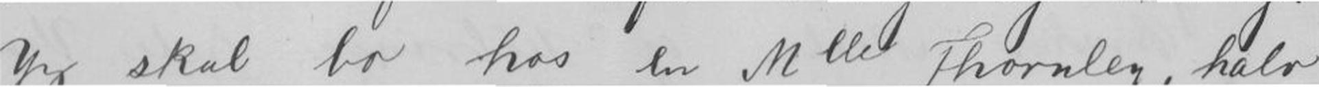Jeg skal bo hos en Mlle Thornley, halv
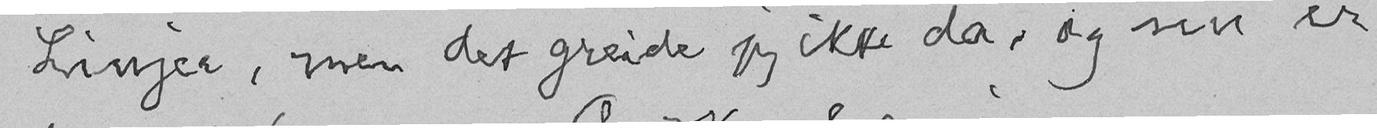Linjer, men det greide jeg ikke da, og nu er
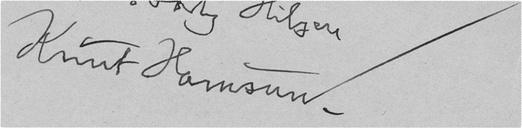Knut Hamsun.
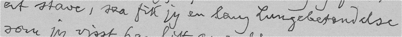at stave, saa fik jeg en lang Lungebetændelse
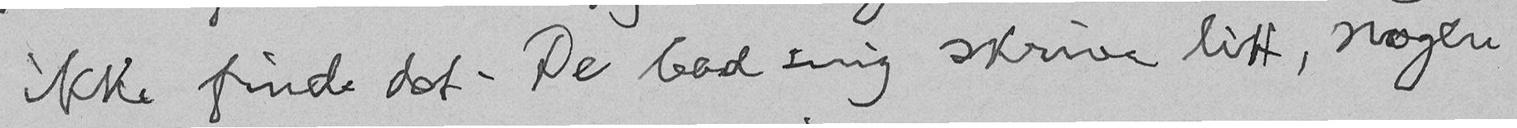ikke finde det. De bad mig skrive litt, nogen
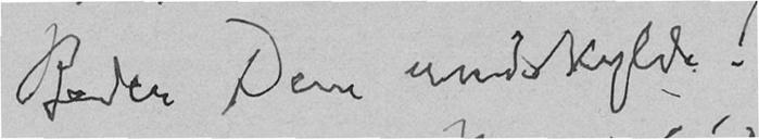Beder Dem undskylde!
